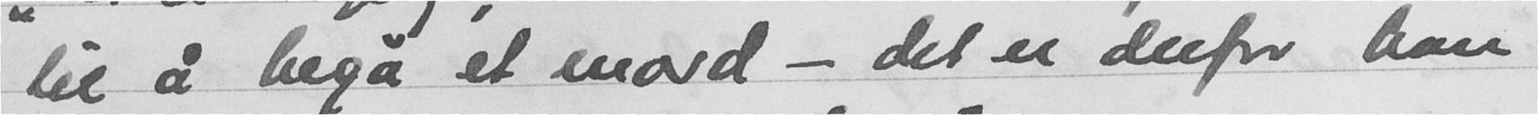til å begå et mord - det er derfor han
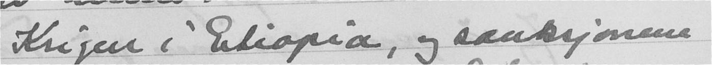Krigen i Etiopia, og sanksjonene
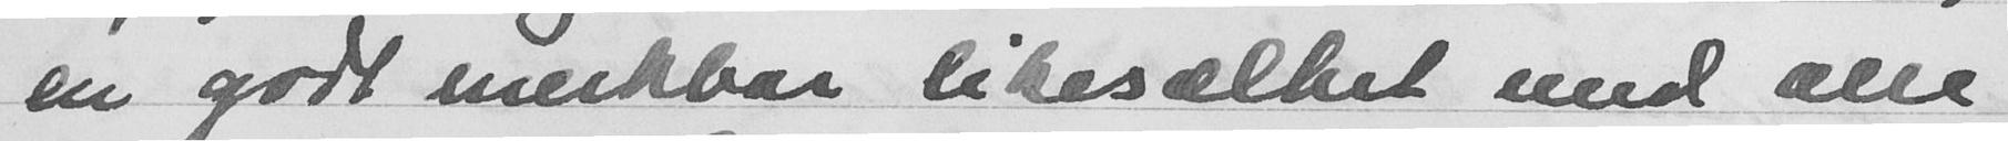en godt merkbar likesælhet med alle
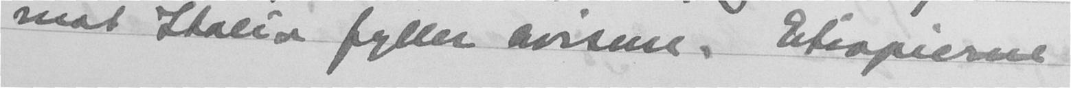mot Italia fyller avisene. Etioperne
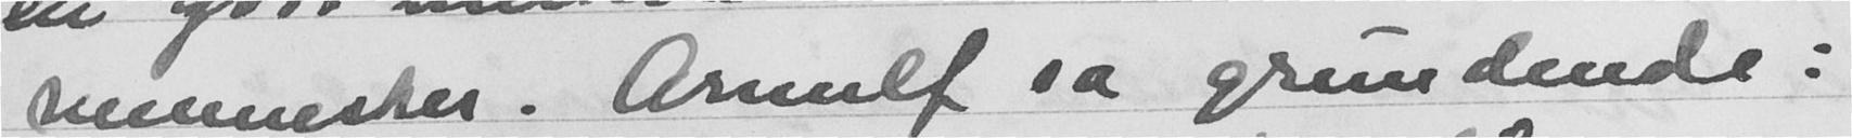mennesker. Arnulf sa grundende:
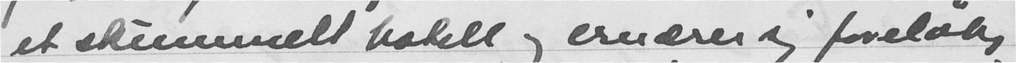et skummelt hotell og ernærer sig foreløbig
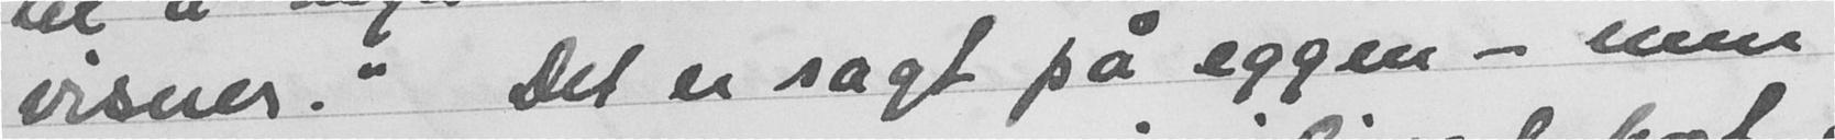visner." Det er sagt på eggen - men
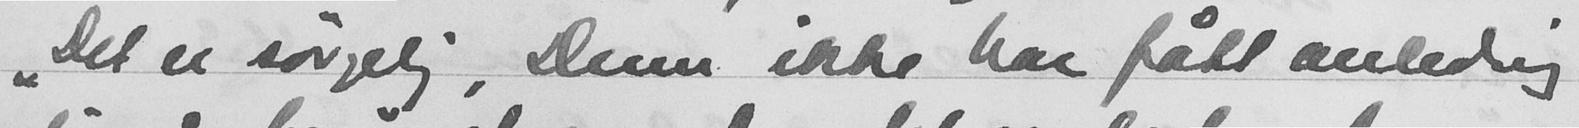"Det er sørgelig, Duun ikke har fått anledning
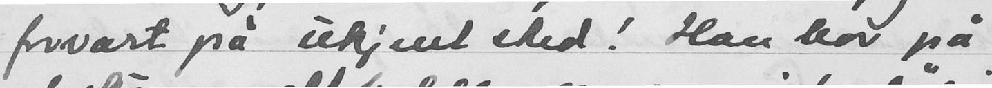forvart på ukjent sted! Han bor på
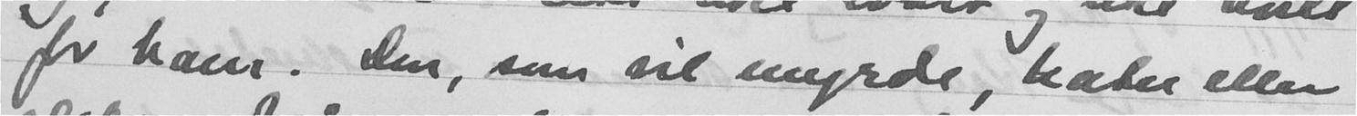for ham. Den, som vil myrde, hater eller
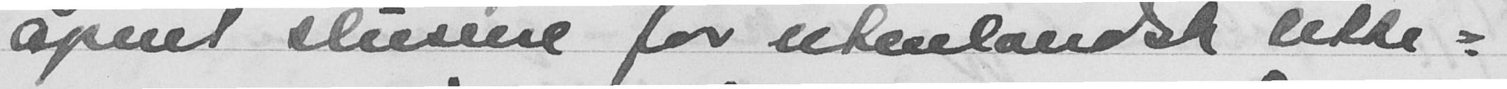åpnet slusene for utenlandsk litte-
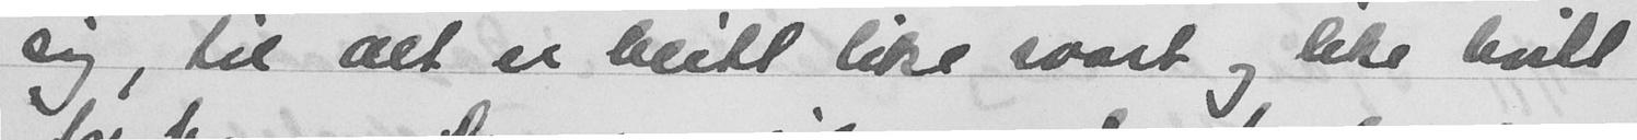sig, til alt er blitt like svart og like hvitt
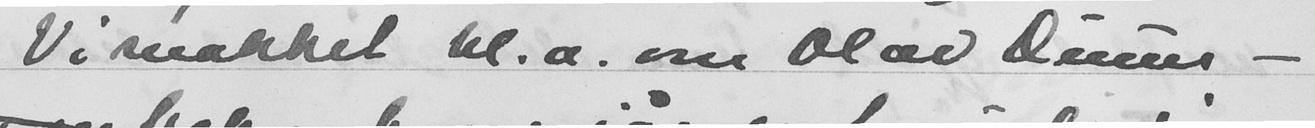Vi snakket bl. a. om Olav Duun -
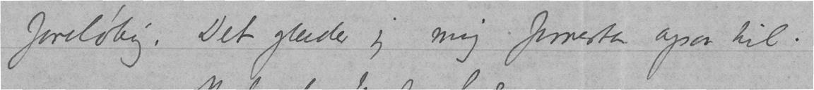foreløbig. Det glæder jeg mig forresten ogsaa til.
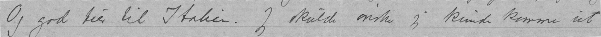Og god tur til Italien. Jeg skulde ønske jeg kunde komme ut
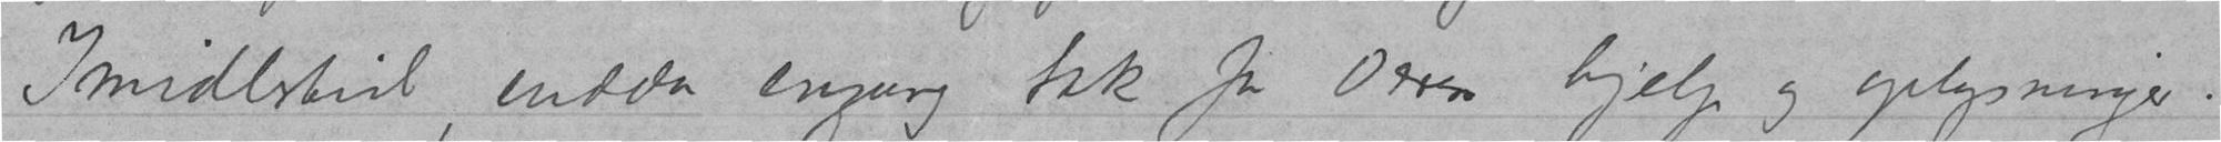Imidlertid endda engang tak for Deres hjelp og oplysninger.
